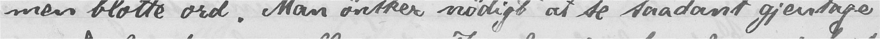men blotte ord. Man ønsker nødigt at se saadant gjentage
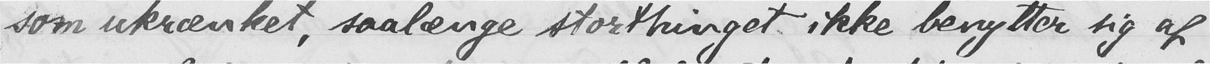som ukrænket, saalænge storthinget ikke benytter sig af
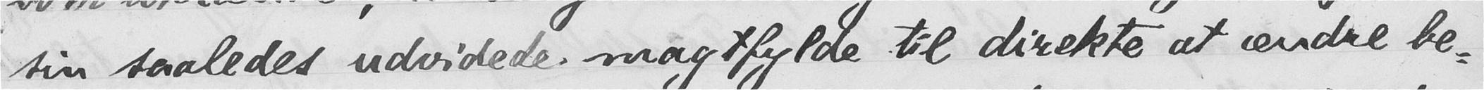sin saaledes udvidede magtfylde til direkte at ændre be-
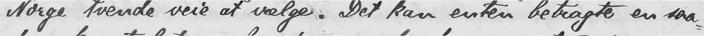Norge tvende veie at vælge. Det kan enten betragte en saa-
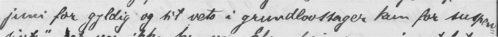juni for gyldig og sit veto i grundlovssager kun for suspen-
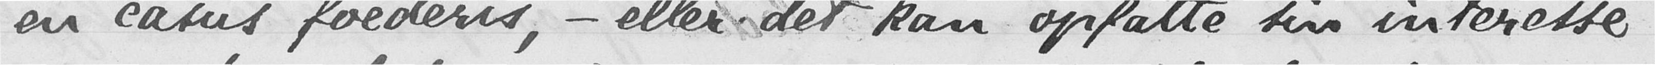en casus foederis, - eller det kan opfatte sin interesse
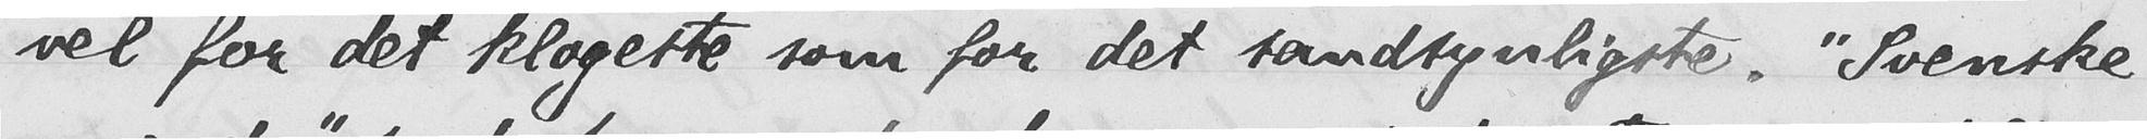vel for det klogeste som for det sandsynligste. "Svenske
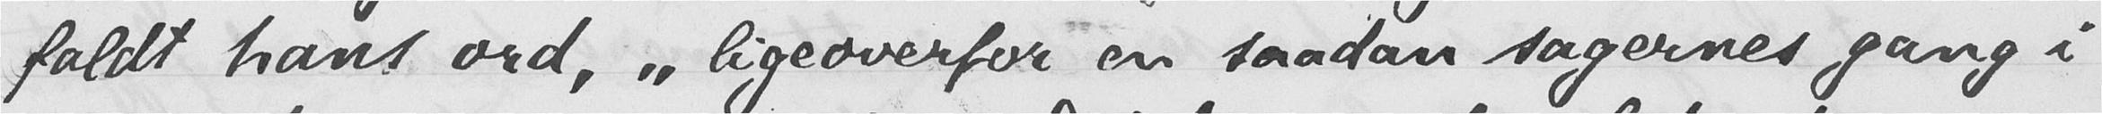faldt hans ord, "ligeoverfor en saadan sagernes gang i
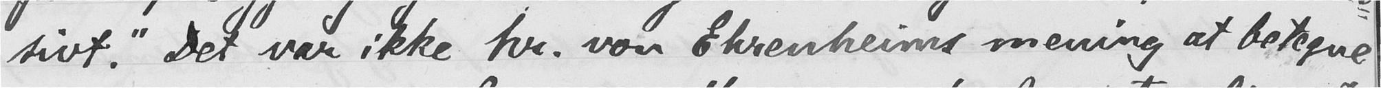sivt." Det var ikke hr. von Ehrenheims mening at betegne
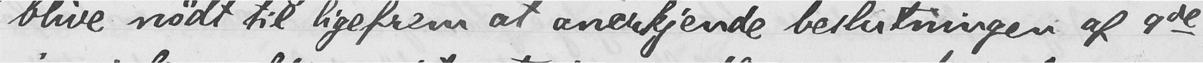blive nødt til ligefrem at anerkjende beslutningen af 9de
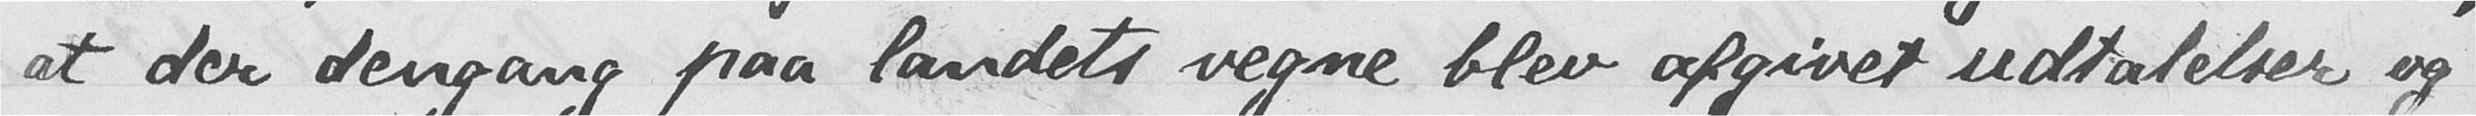at der dengang paa landets vegne blev afgivet udtalelser og
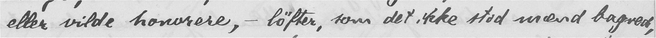eller vilde honorere, - løfter, som det ikke stod mænd bagved,
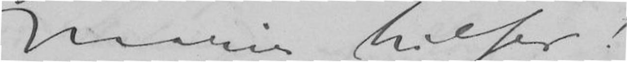Marie hilser!
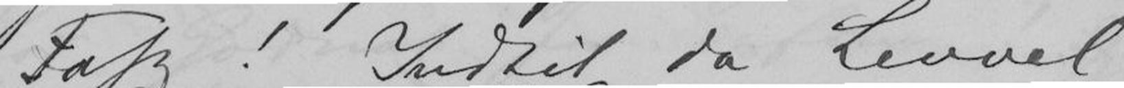Fass! Indtil da Levvel!
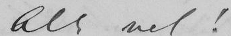Alt vel!
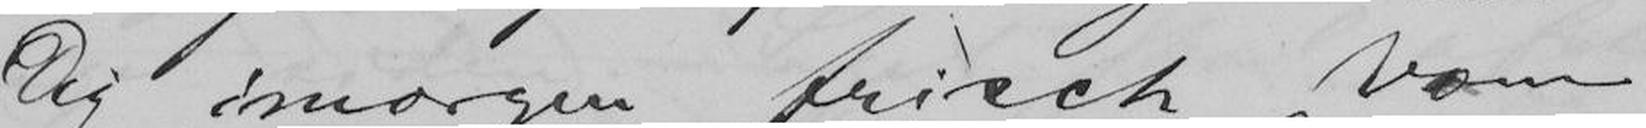Dig imorgen frisch vom
